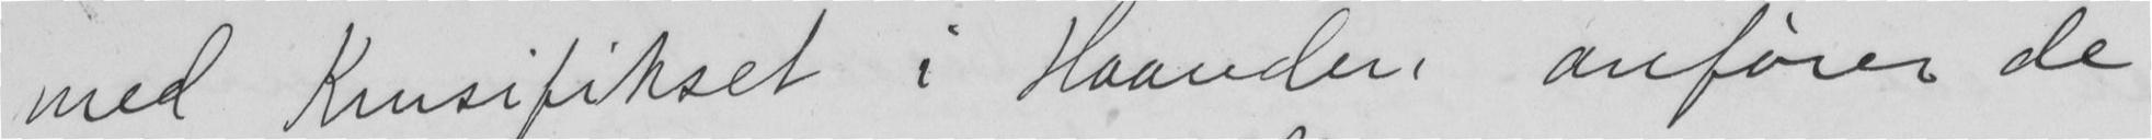med Krusifikset i Haanden anfører de
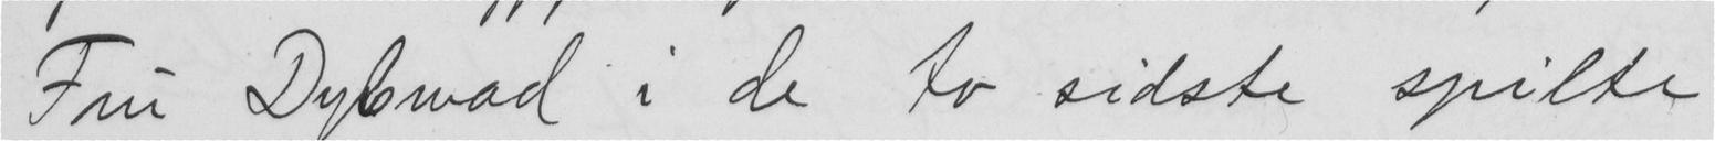Fru Dybwad i de to sidste spilte
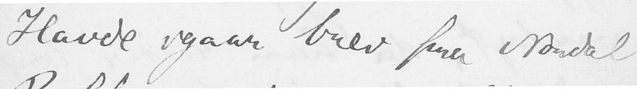Havde igaar brev fra Nordal
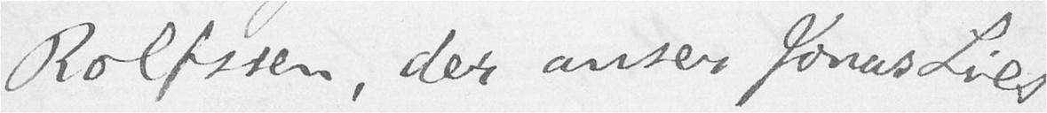Rolfssen, der anser Jonas Lies
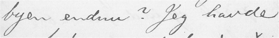byen endnu? Jeg havde
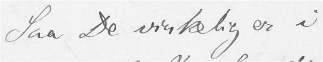Saa De virkelig er i
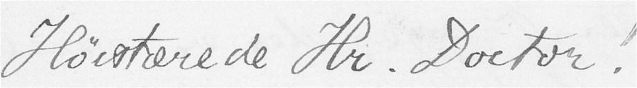Høistærede Hr. Doctor!
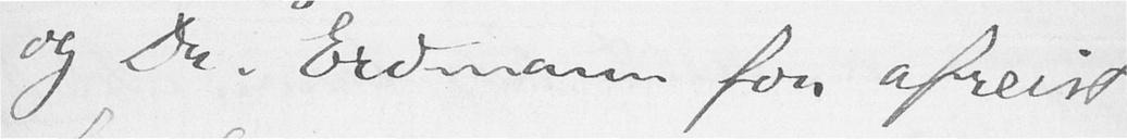og Dr. Erdmann for afreist
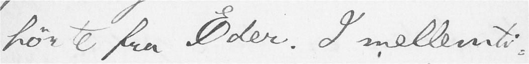hørte fra Eder. I mellemti-
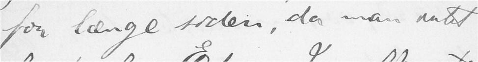for længe siden, da man intet
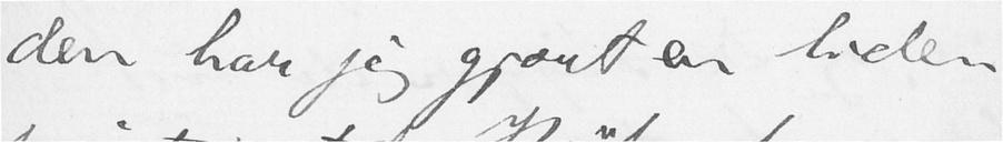den har jeg gjort en liden
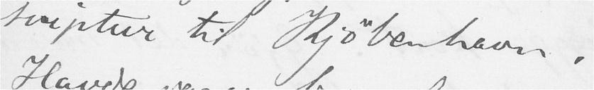sviptur til Kjøbenhavn.
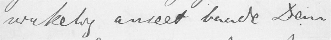virkelig anseet baade Dem
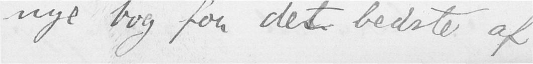nye bog for det bedste af
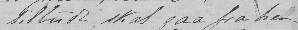tilbudt, skal gaa fra hen-
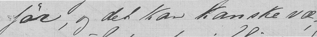før, og det kan kanske væ-
